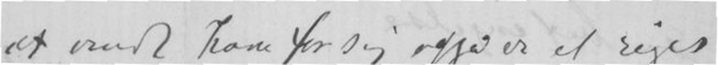at vende ham for sig også er et riges
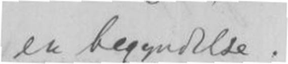en begyndelse.
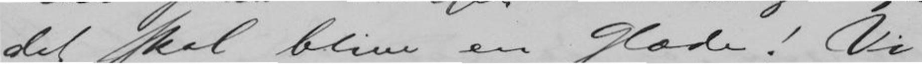det skal blive en Glæde! Vi
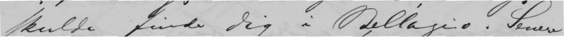skulde finde Dig i Bellagio. Senere
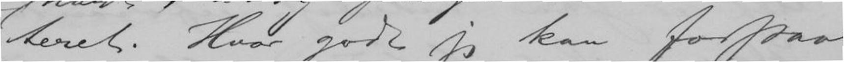teret. Hvor godt jeg kan forstaa
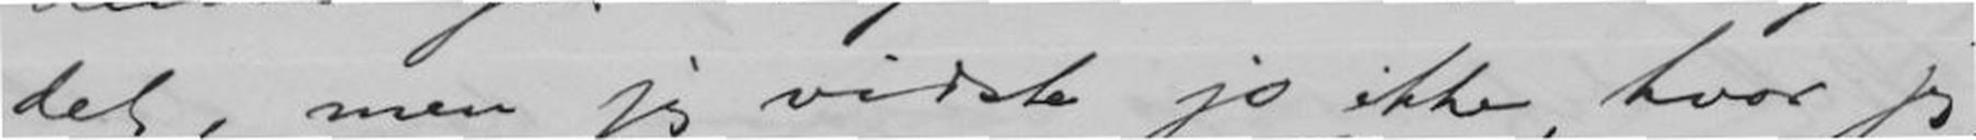det, men jeg vidste jo ikke, hvor jeg
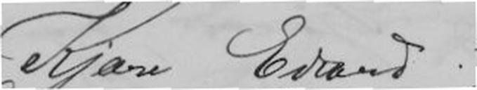Kjære Edvard!
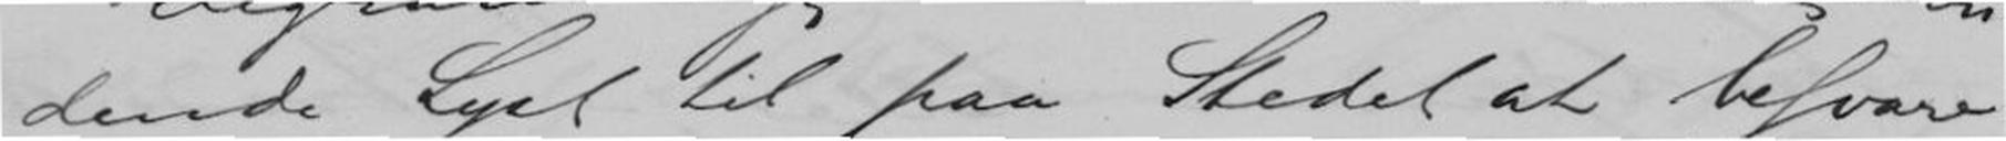dende Lyst til paa Stedet at besvare
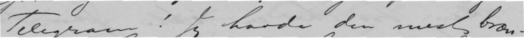Telegram! Jeg havde der mest bræn-
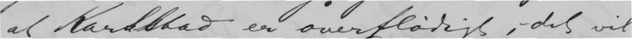at Karlsbad er overflødigt; det vil
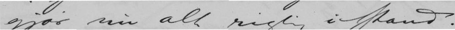gjør nu alt rigtig istand!
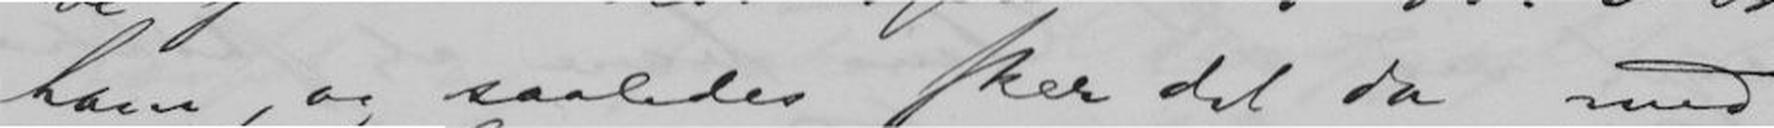ham, og saaledes sker det da med
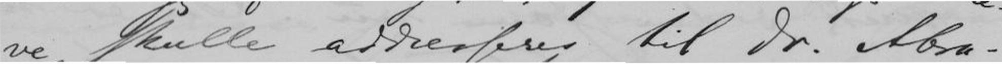ve skulde addresseres til Dr. Abra-
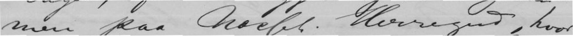men paa Næsset. Herregud, hvor
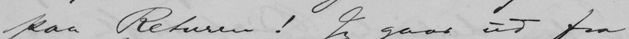paa Returen! Jeg Gaar ud fra,
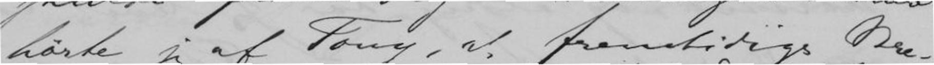hørte jeg af Tony, at fremtidige Bre-
